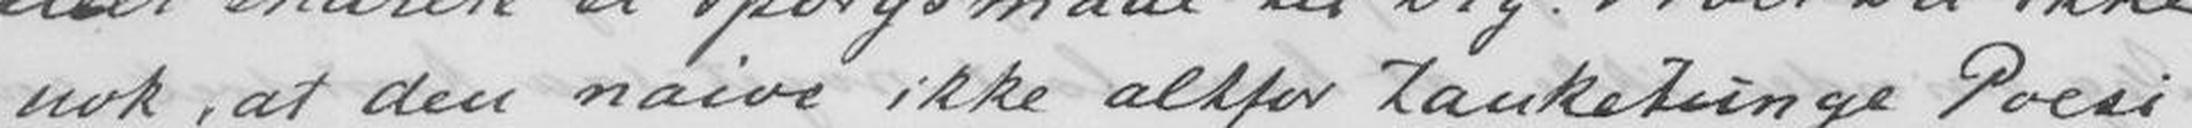nok, at den naive ikke altfor tanketunge Poesi
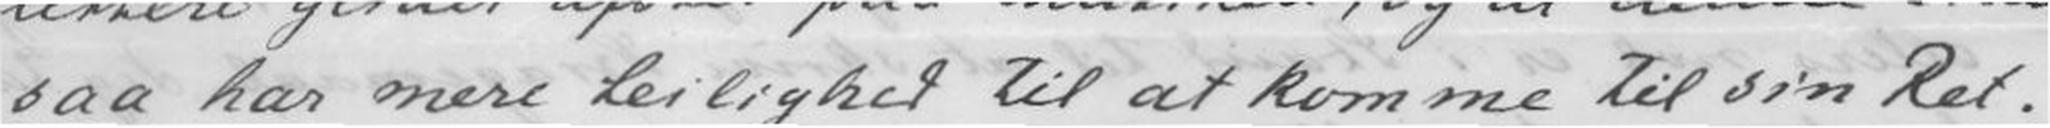saa har mere Leilighed til at komme til sin Ret.
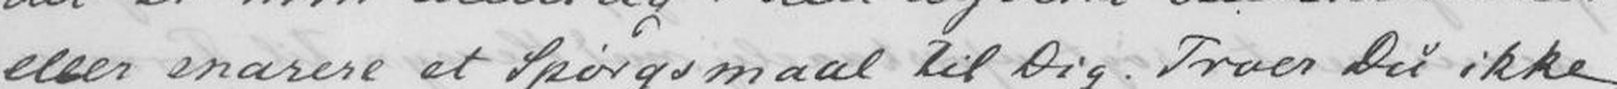eller snarere et Spørgsmaal til Dig. Troer Du ikke
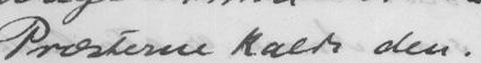Præsterne kalde den.
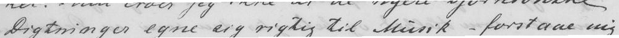Digtninger egne sig rigtig til Musik - forstaae mig
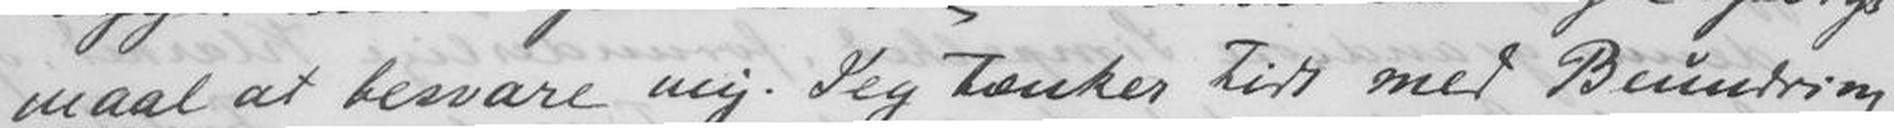maal at besvare mig. Jeg tænker Tidt med Beundring
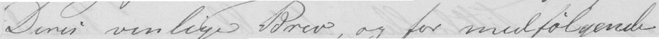Deres venlige Brev, og for medfølgende
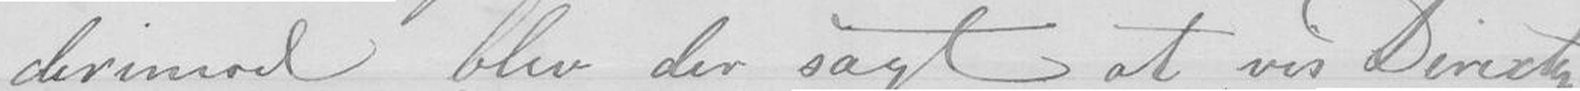derimod blev der sagt at vis Directøren
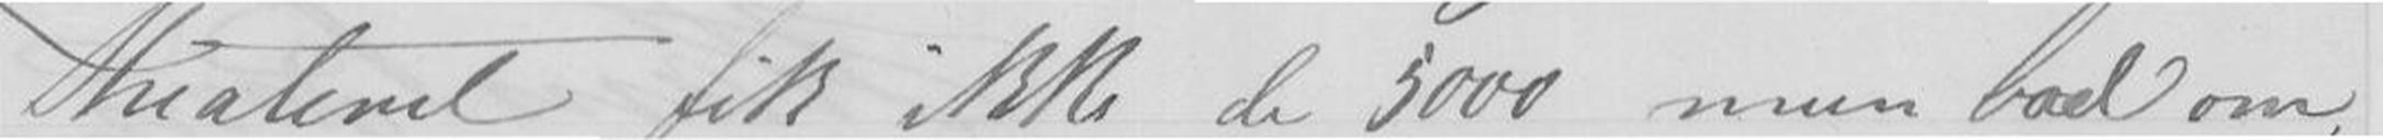Theateret fik ikke de 5000 man bad om,
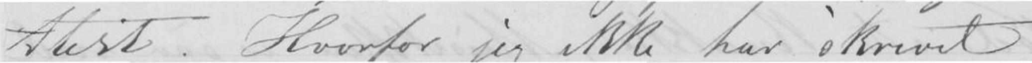Atest. Hvorfor jeg ikke har skrevet
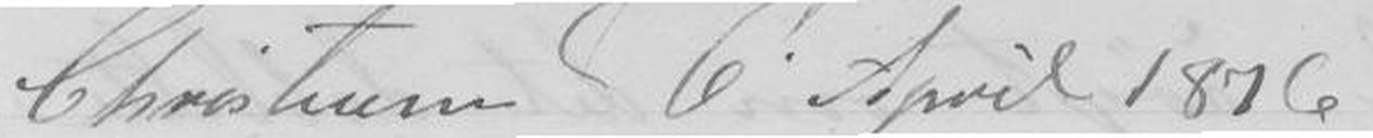Christiania 6t April 1876
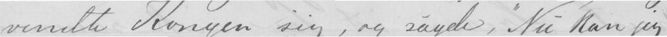vendte Kongen sig, og sagde, "Nu kan jeg
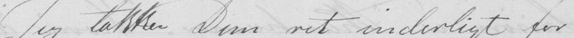Jeg takker Dem ret inderligt for
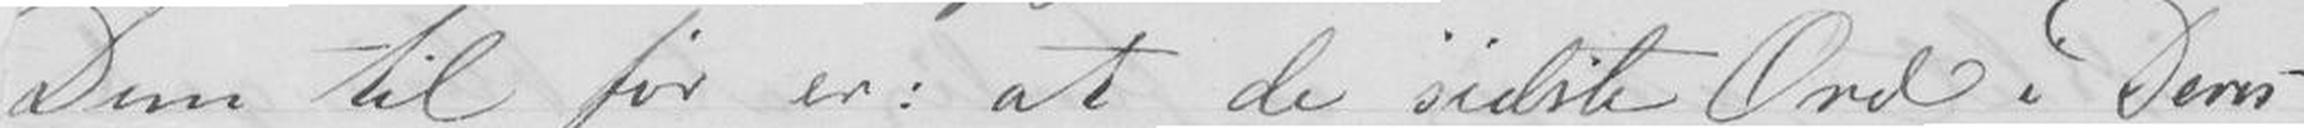Dem til før er: at de sidste Ord i Deres
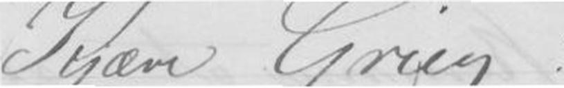Kjære Grieg
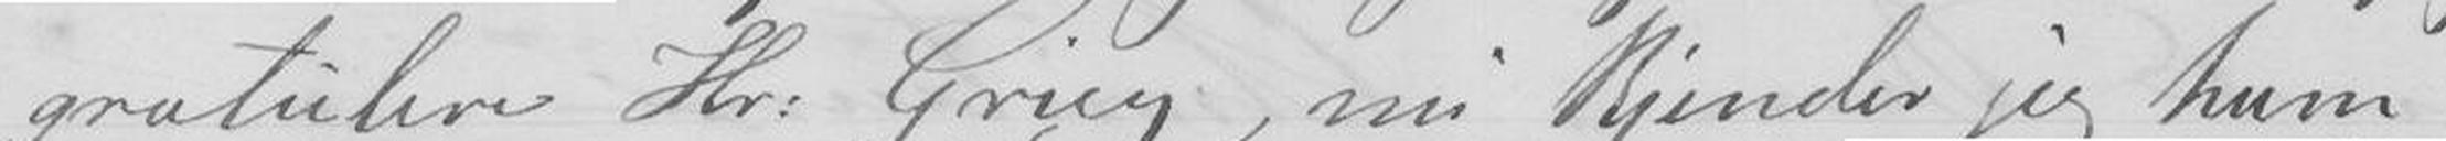gratulere Hr: Grieg, nu kjender jeg ham
